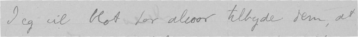Jeg vil blot for alvor tilbyde dem, at
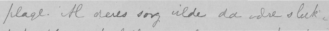plage. Al deres sorg vilde da være sluk-
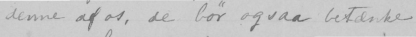denne af os, de bør ogsaa betænke,
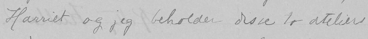Harriet og jeg beholder disse to ateliers
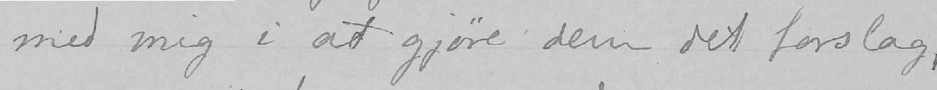med mig i at gjøre dem det forslag,
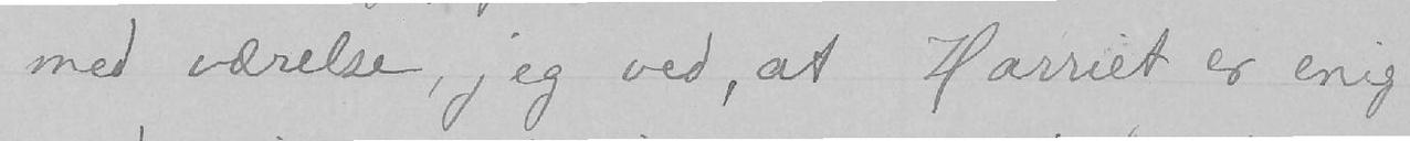med værelse, jeg ved, at Harriet er enig
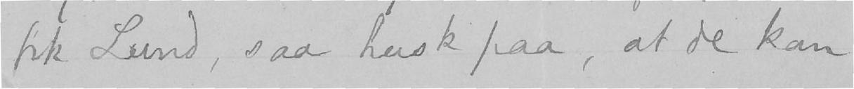frk Lund, saa husk paa, at de kan
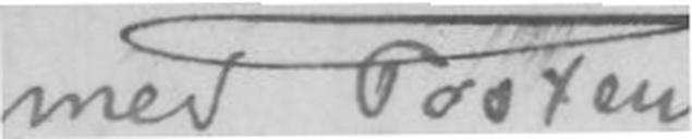med Posten.
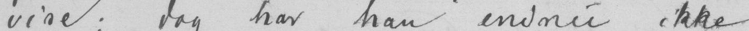vise; Dog har han endnu ikke
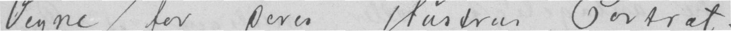Vegne for Deres Hustrus Portræt.
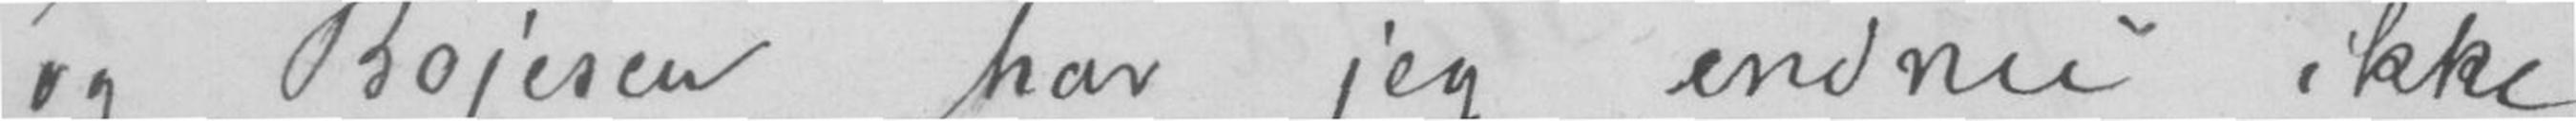og Bojesen har jeg endnu ikke
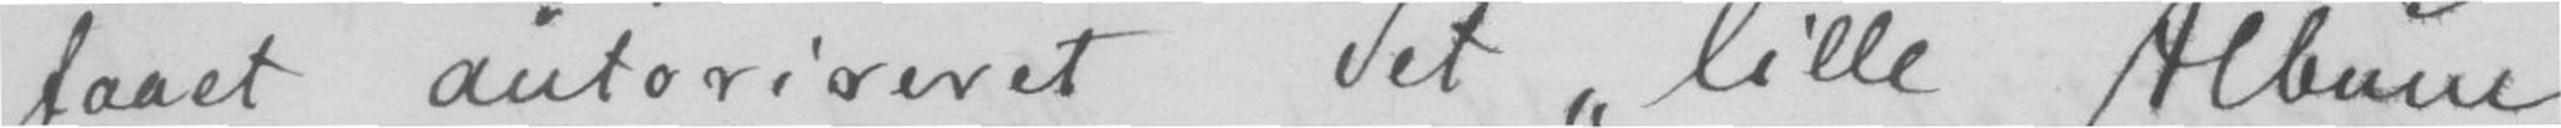faaet autoriseret det "lille Almune
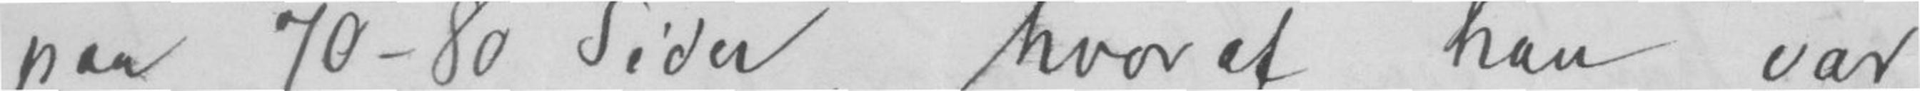paa 70-80 sider, hvoraf han var
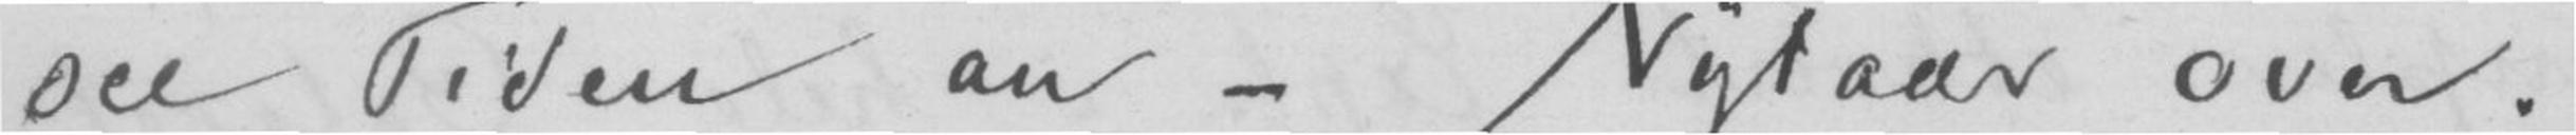see Tiden an - Nytaar over.
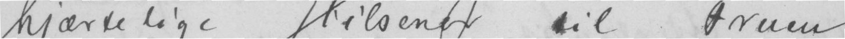hjærtelige Hilsener til Fruen
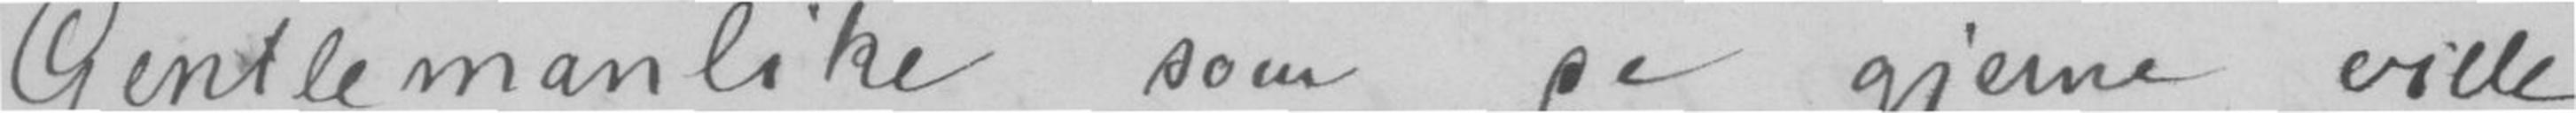Gentleman like som de gjerne ville
